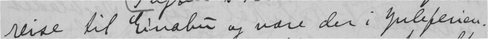reise til Einabu og være der i Juleferien.
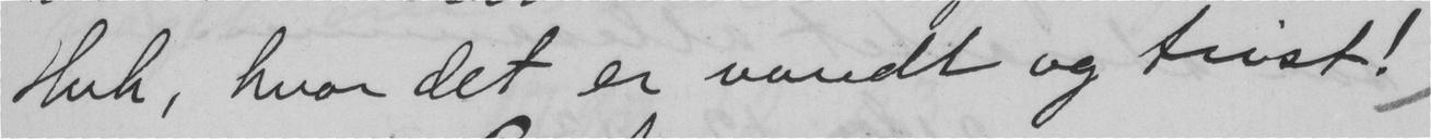Huh, hvor det er vondt og trist!
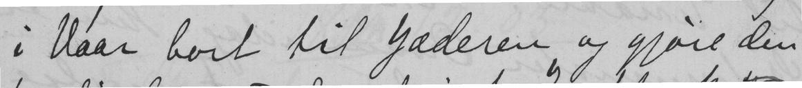i Vaar bort til Jæderen og gjøre den
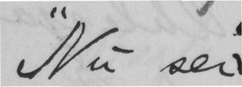"Nu ser
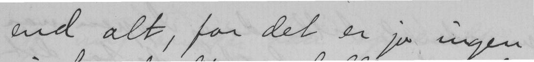end alt, for det er jo ingen
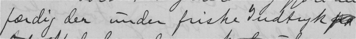færdig der under friske Indtryk
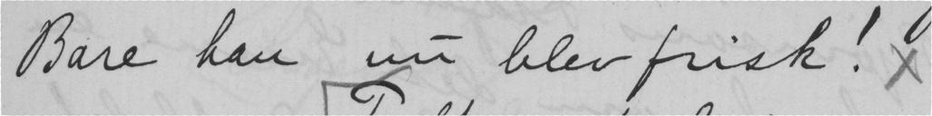Bare han nu blev frisk! x
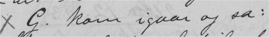x G. kom igaar og sa:
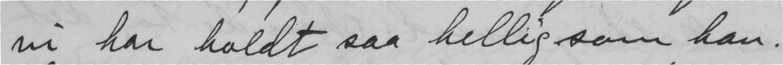vi har holdt saa hellig som han.
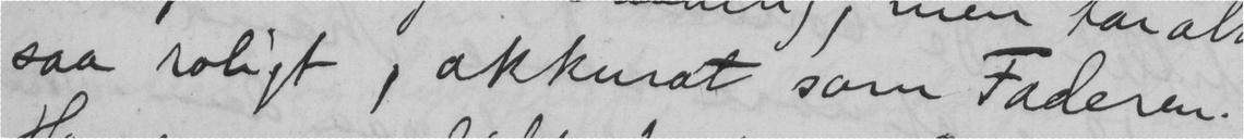saa roligt, akkurat som Faderen.
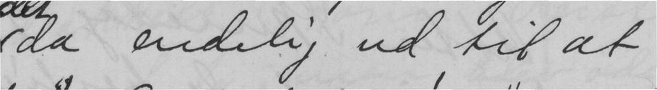da endelig ud til at
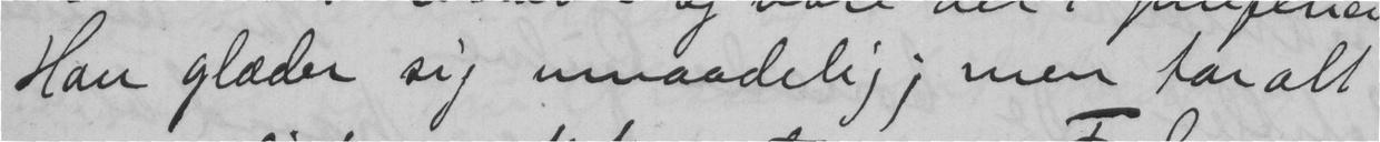Han glæder sig umaadelig; men tar alt
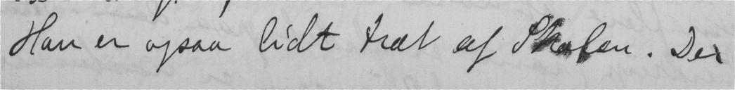Han er ogsaa lidt træt af Skolen. Der
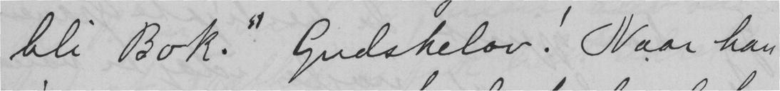bli Bok." Gudskelov! Naar han
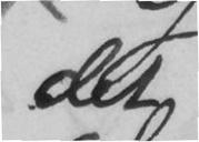det
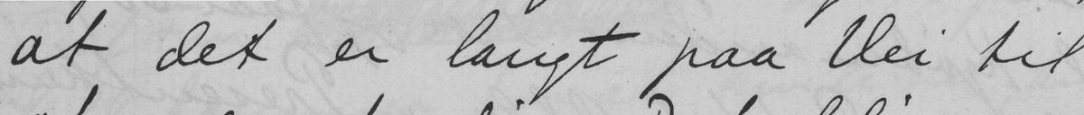at det er langt paa vei til
